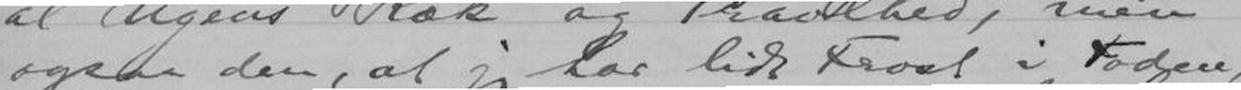ogsaa den, at jeg har lidt Frost i Foden,
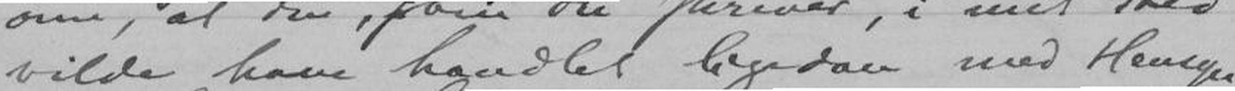vilde have handlet ligedan med Hensyn
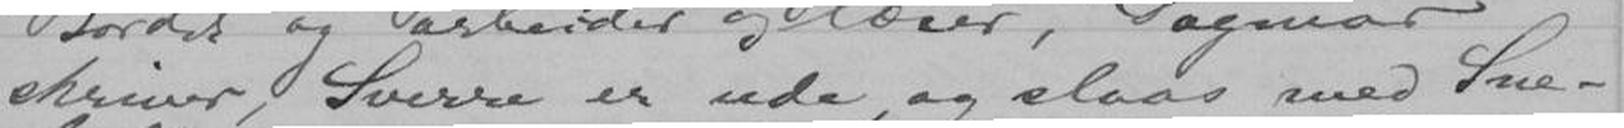skriver, Sverre er ude og slaas med Sne-
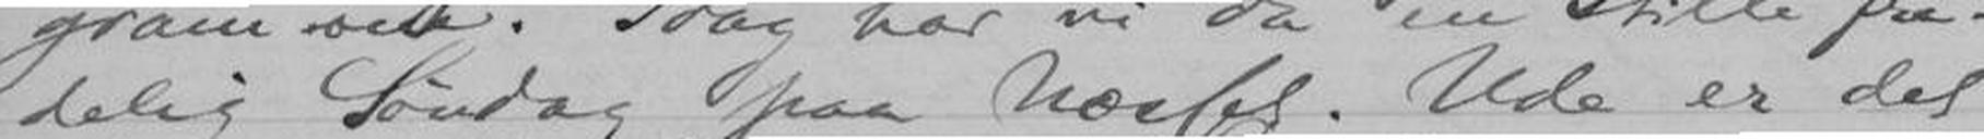delig Søndag paa Næsset. Ude er det
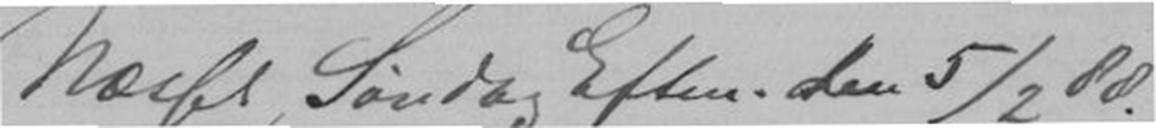Næsset, Søndag Eftem. den 5/2 88
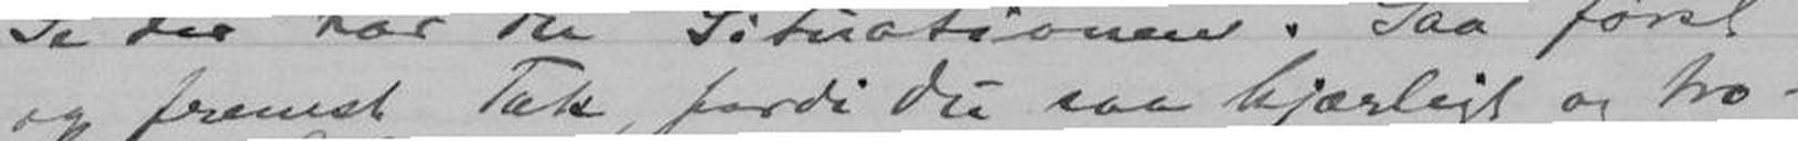og fremst Tak, fordi Du saa kjærligt og tro-
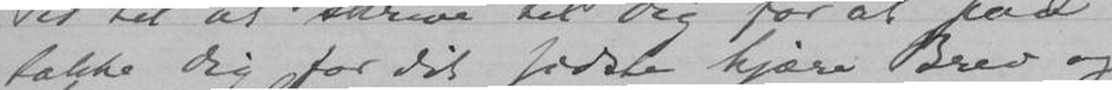takke dig for dit sidste kjære Brev og
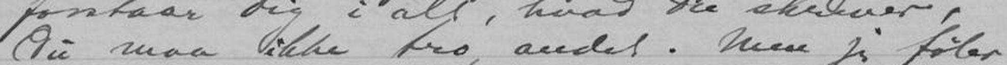Du maa ikke tro andet. Men jeg føler
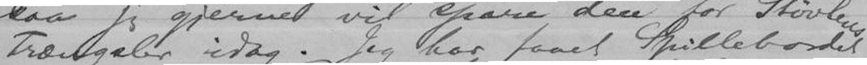Trængsler idag. Jeg har faaet Spillebordet
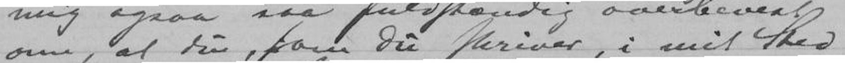om, at Du, som Du skriver, i mit Sted
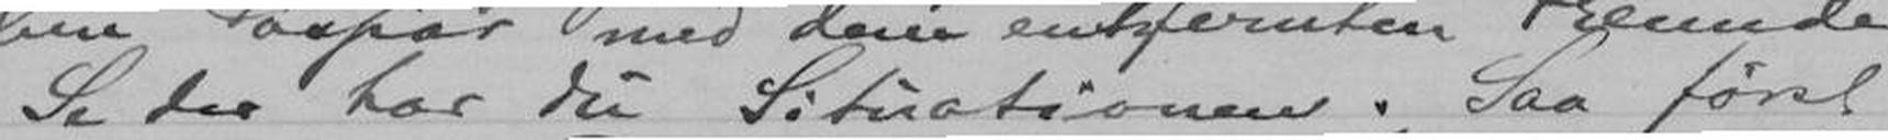Se der har Du situationen. Saa først
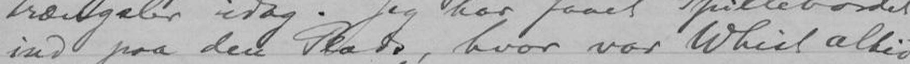ind paa den Plads, hvor vor Whist altid
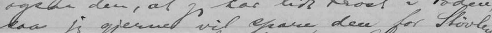saa jeg gjerne vil spare den for Støvlens
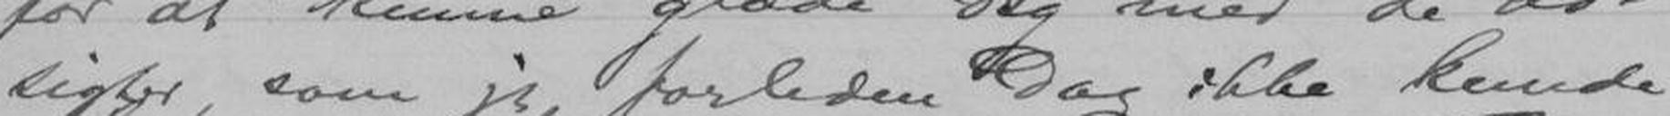sigter, som jeg forleden Dag ikke kunde
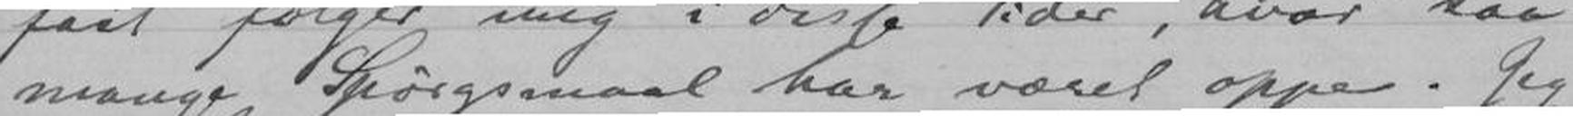mange Spørgsmaal har været oppe. Jeg
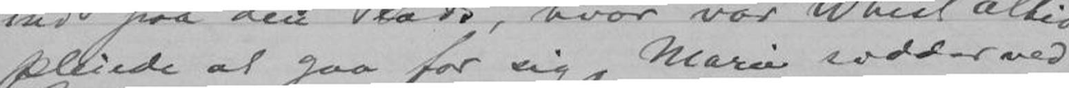pleiede at gaa for sig, Marie sidder ved
